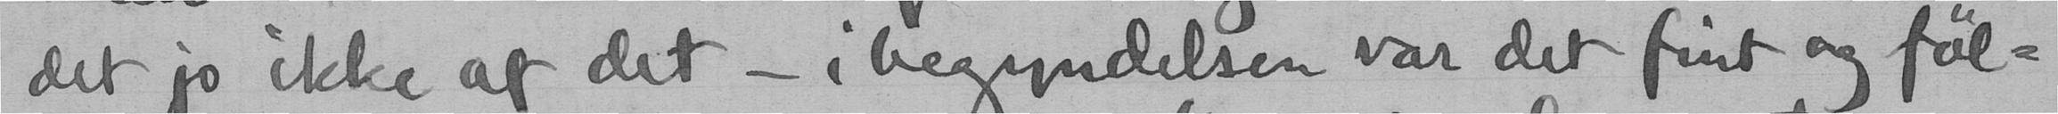det jo ikke af det - i begyndelsen var det fint og føl-
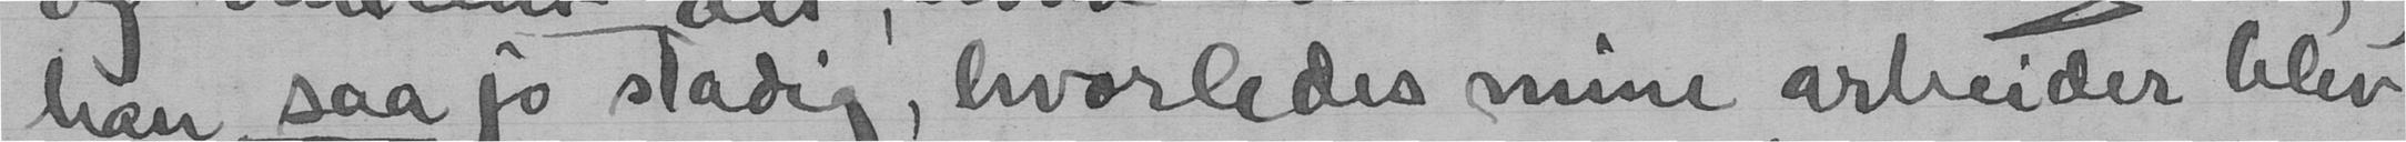han saa jo stadig, hvorledes mine arbreider blev
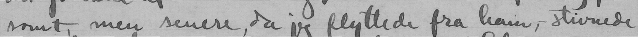somt, men senere da jeg flyttede fra ham, -stivnede
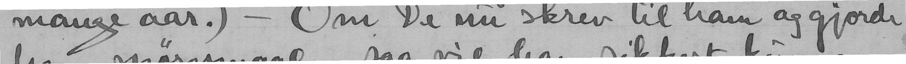mange aar.) - Om De nu skrev til ham og gjorde
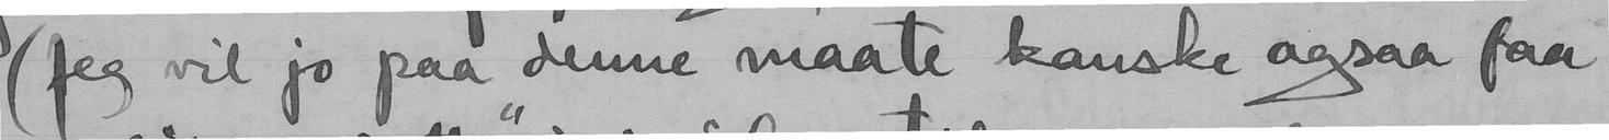(Jeg vil jo paa denne maate kanske ogsaa faa
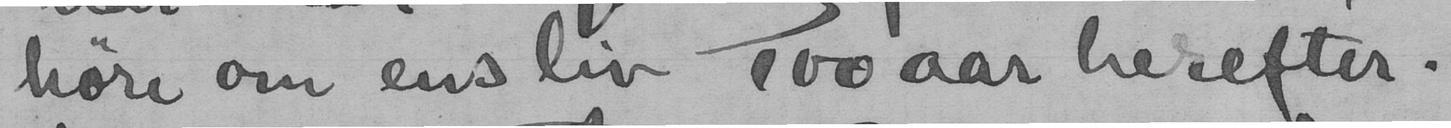høre om ens liv 100 aar herefter.
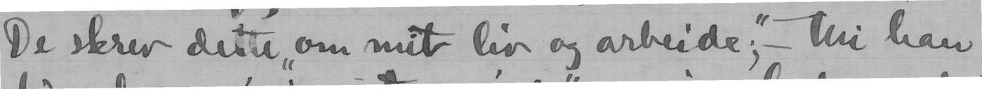De skrev dette "om mit liv og arbeide"; - thi han
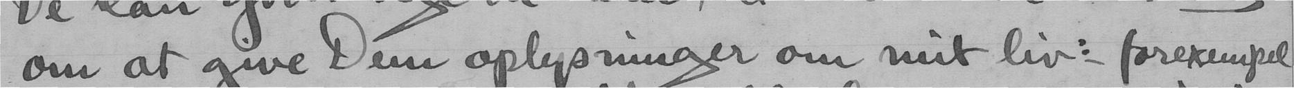om at give Dem oplysninger om mit liv :- forexempel
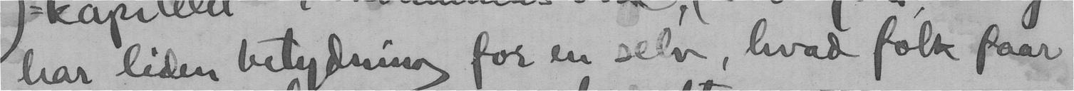har liden betydning for en selv, hvad folk faar
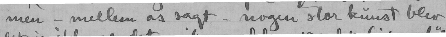men - mellem os sagt - nogen stor kunst blev
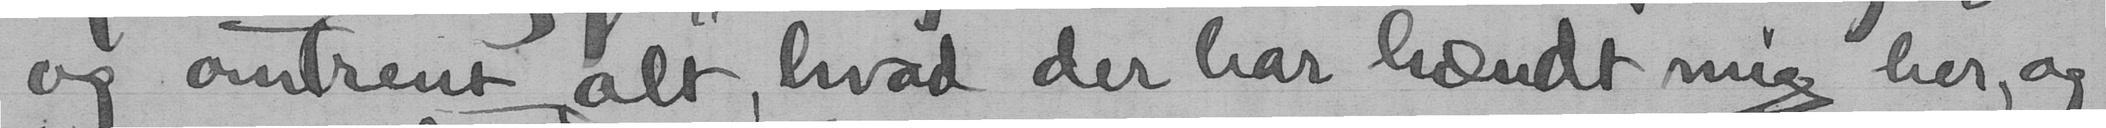og omtrent alt, hvad der har hændt mig her, og
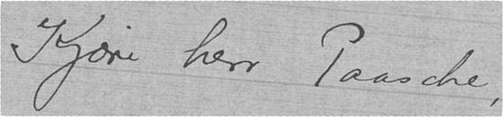Kjære herr Paasche,
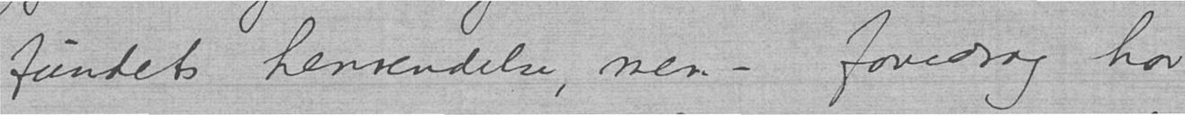fundets henvendelse, men – foredrag har
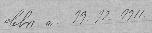Chr. a. 19.12.1911.
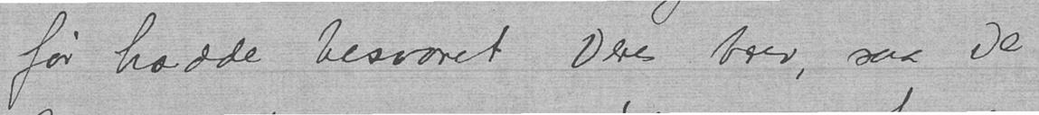før hadde besvaret Deres brev, saa De
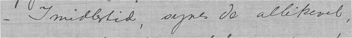- Imidlertid, synes De allikevel,
